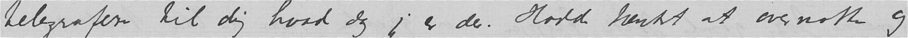telegrafere til dig hvad dag jeg er der. Hadde tænkt at overnatte og
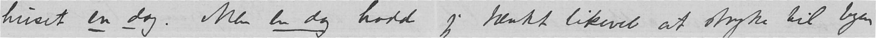huset en dag. Men en dag hadde jeg tenkt likevel at stryke til byen –
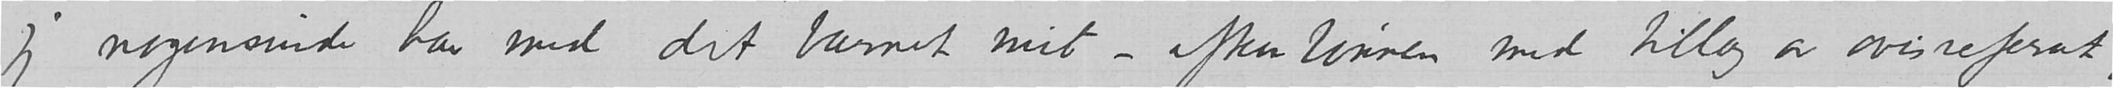jeg nogensinde har med det barnet mit – aftenbønnen
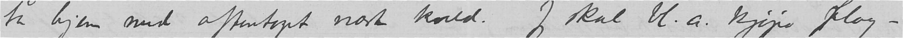ta hjem med aftentoget næste kveld. Jeg skal bl.a. kjøpe flag –
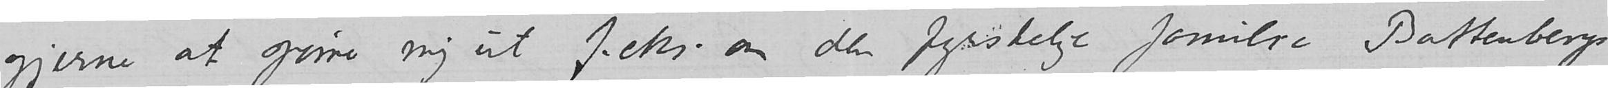gjerne at spørre mig ut f.eks. om den fyrstelige familie Battenbergs forhold
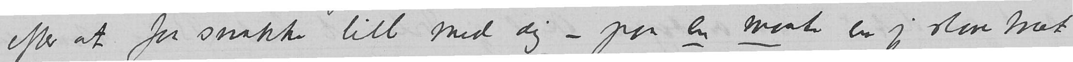efter at faa snakke litt med dig – paa en maate er jeg stor træt
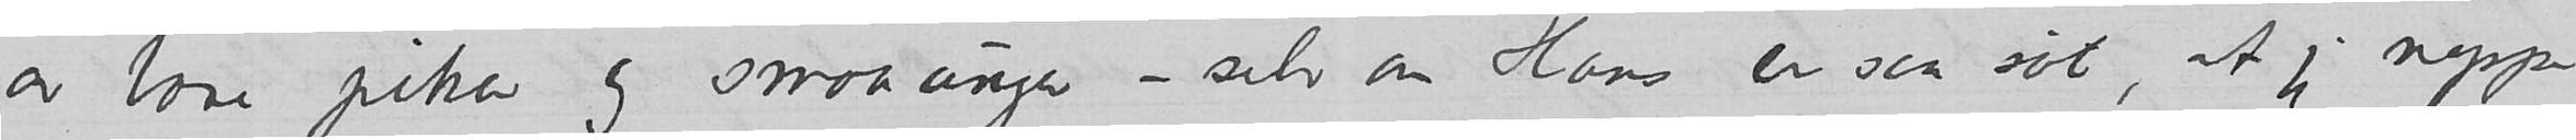av bare piker og smaaunger – selv om Hans er saa søt, at jeg neppe
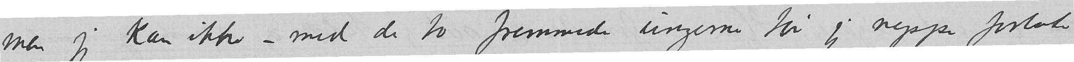men jeg kan ikke – med de to fremmede ungerne tør jeg neppe forlate
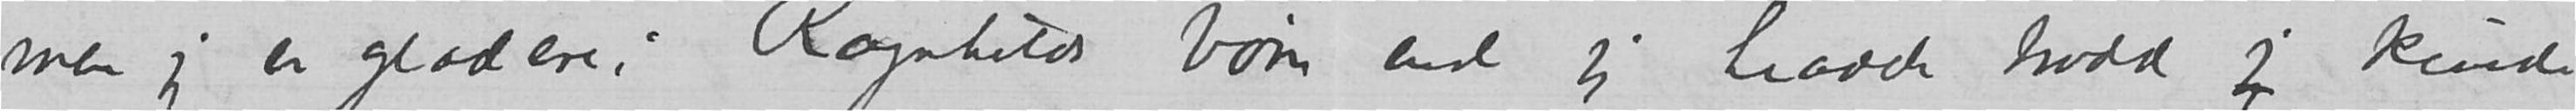men jeg er gladere i Ragnhilds børn end jeg hadde trodd jeg kunde
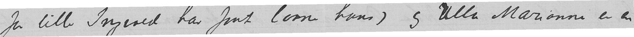for lille Ingvald har faat laane hans) og Ulla Marianne er en
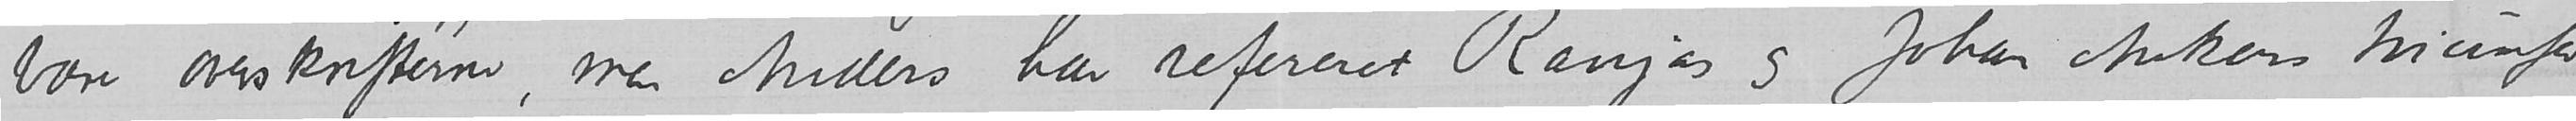bare overskrifterne, men Anders har refereret Ranjas og Johan Ankers triumfer
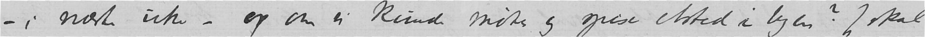i næste uke – og om vi kunde møtes og spise etsted i byen? Jeg skal
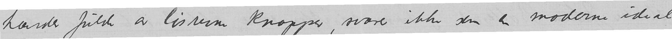hænder fulde av lisrerne kropper, svarer ikke som en moderne ideal
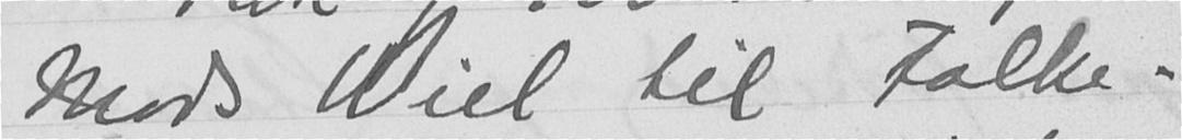Mads Wiel til Folke-
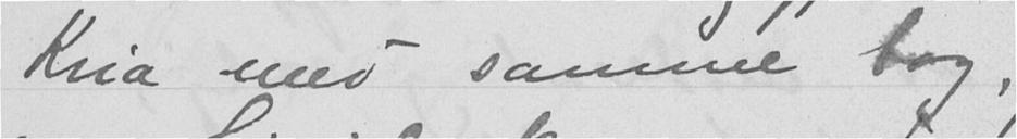Kria med samme tog,
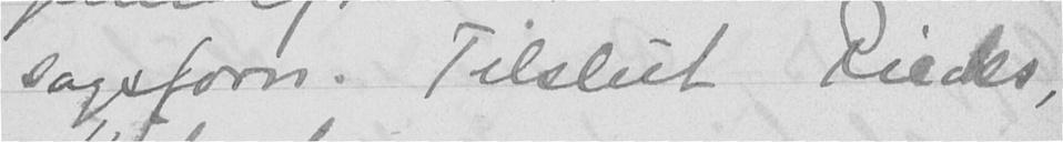sagsforn. Tilslut Riecks,
